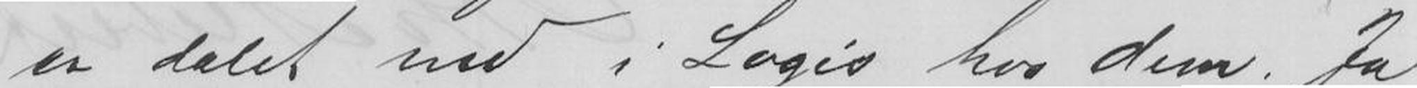er dalet ned i Logis hos dem. Ja
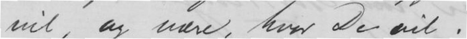vil, og være, hvor De vil!
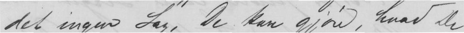det ingen Sag, De kan gjøre, hvad De
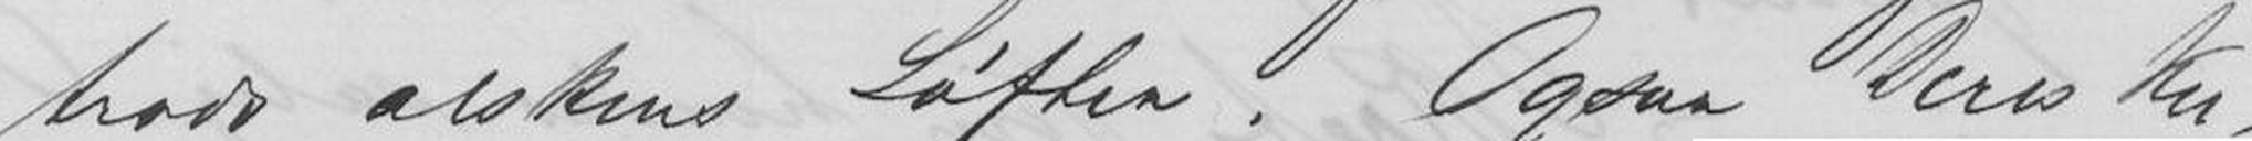trods alskens Løfter. Ogsaa Deres Ku-
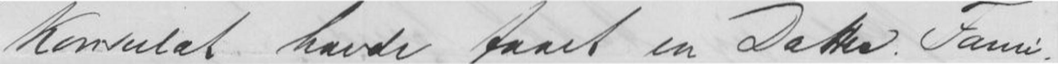Konsulat havde faaet en Datter. Fami-
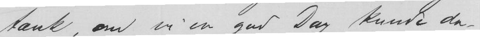tænk, om vi en god Dag kunde da-
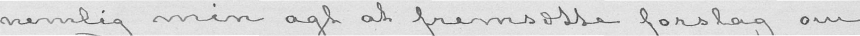nemlig min agt at fremsætte forslag om
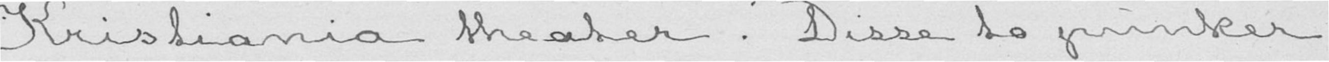Kristiania theater: Disse to punker
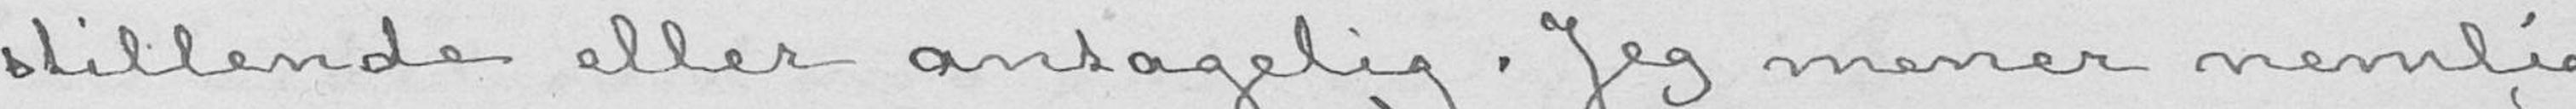stillende eller antagelig. Jeg mener nemlig
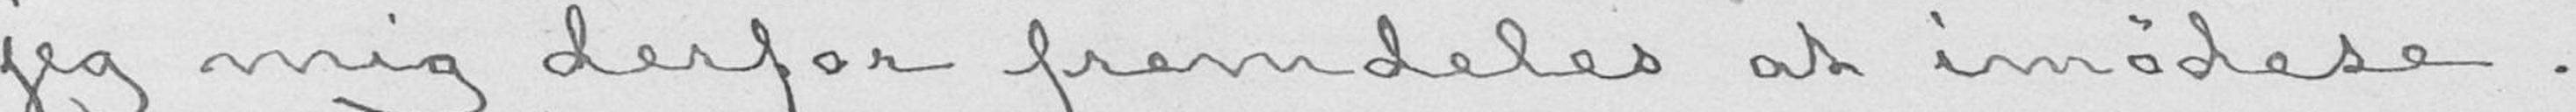jeg mig derfor fremdeles at imødese.
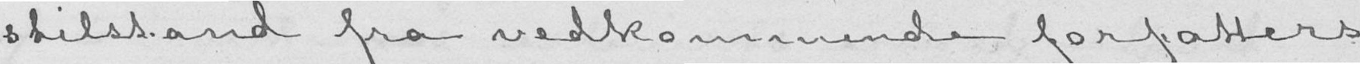stilstand fra vedkommende forfatters
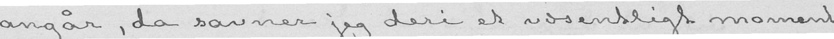angår, da savner jeg deri et væsentligt moment
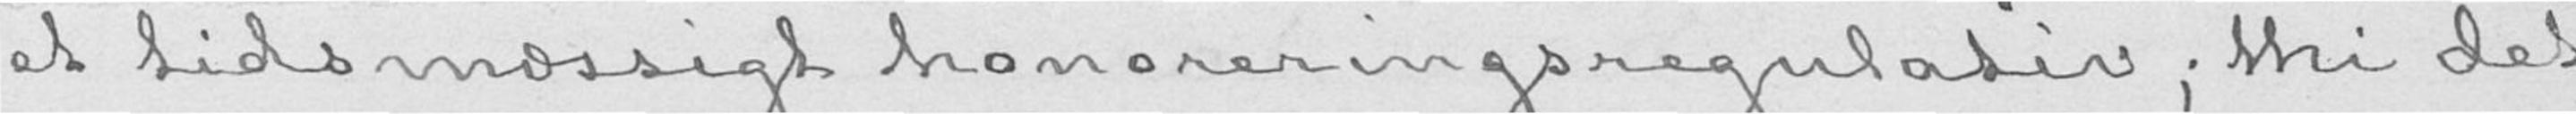et tidsmæssigt honoreringsregulativ; thi det
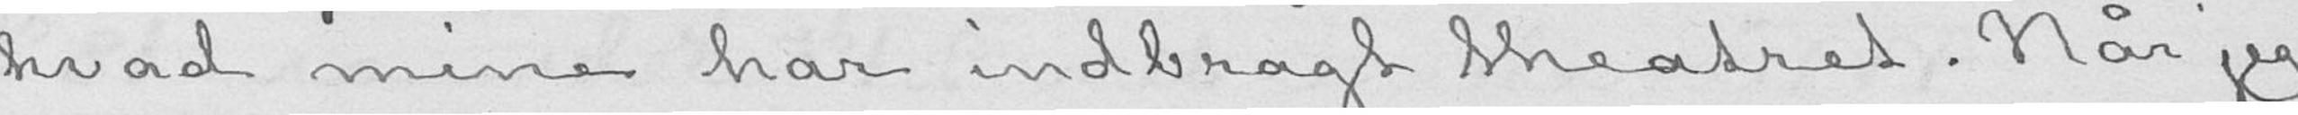hvad mine har indbragt theatret. Når jeg
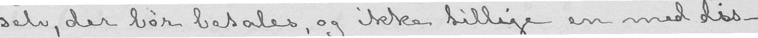selv, der bør betales, og ikke tillige en med dsis-
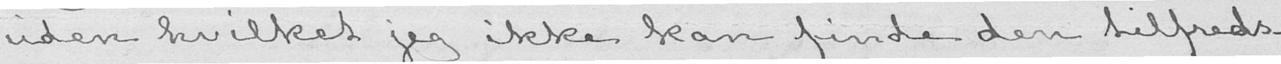uden hvilket jeg ikke kan finde den tilfreds-
